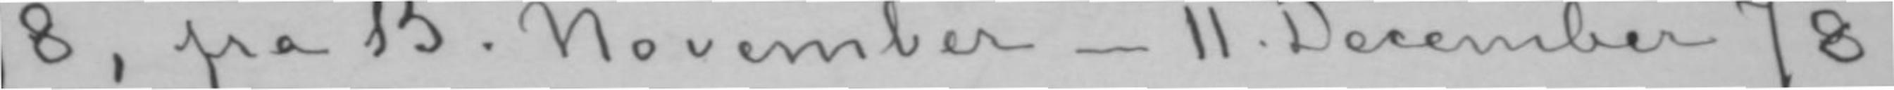78, fra 15. November- 11. December 78,
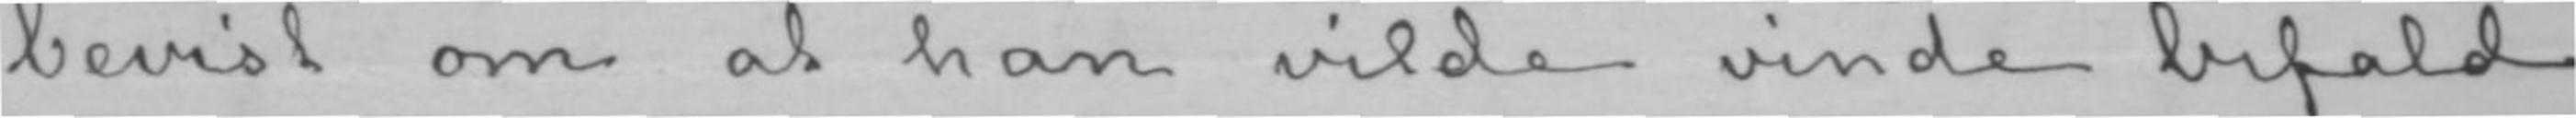bevist om at han vilde vinde bifald
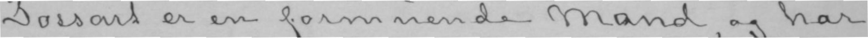Possart er en formuende Mand, og har
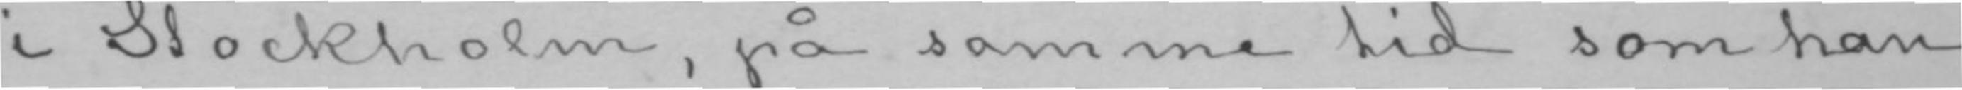i Stockholm, på samme tid som han
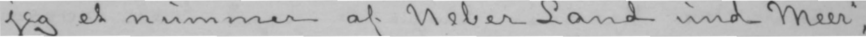jeg et nummer af «Ueber Land und Meer»,
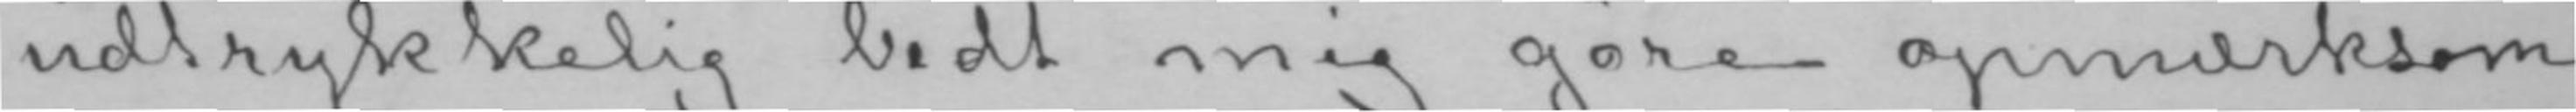udtrykkelig bedt mig gøre opmærksom
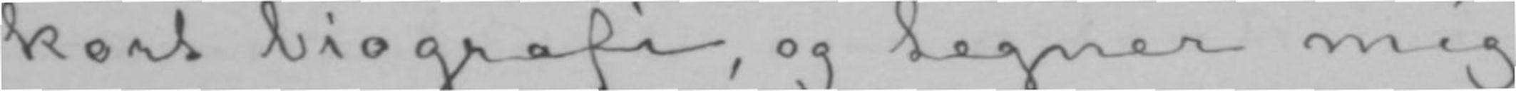kort biografi, og tegner mig
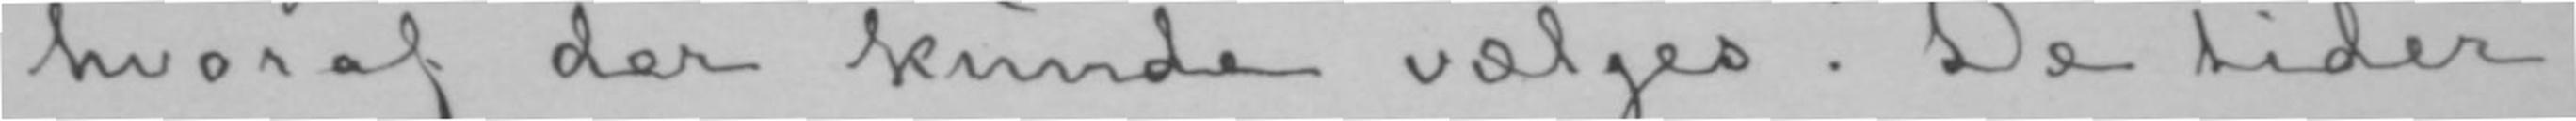hvoraf der kunde vælges. De tider,
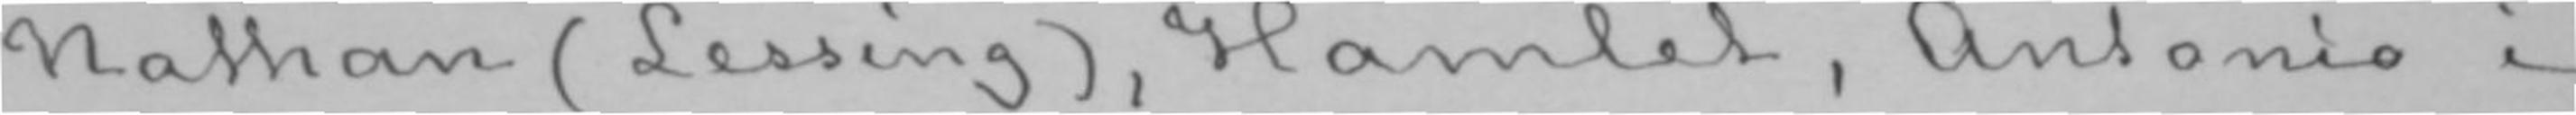Nathan (Lessing), Hamlet, Antonio i
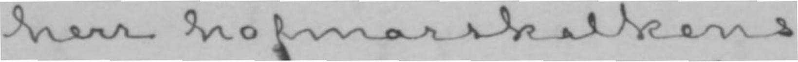herr hofmarskalkens
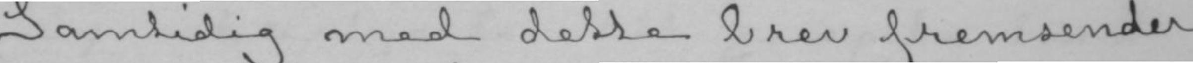Samtidig med dette brev fremsender
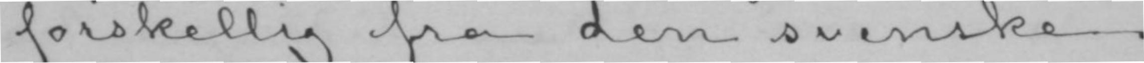forskellig fra den svenske.
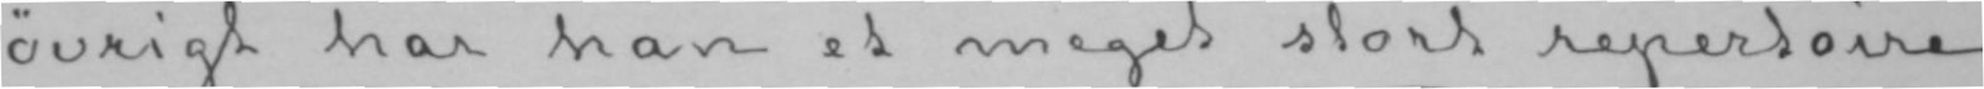øvrigt har han et meget stort repertoire,
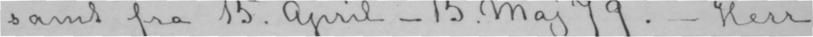samt fra 15. April- 15. Maj 79.- Herr
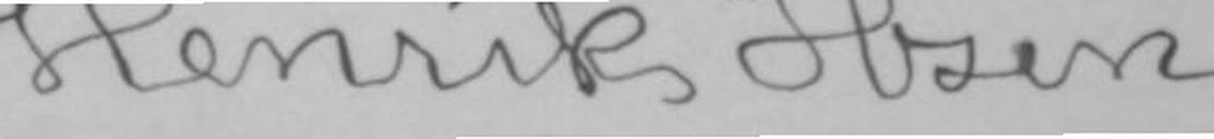Henrik Ibsen.
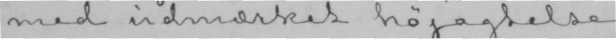med udmærket højagtelse
